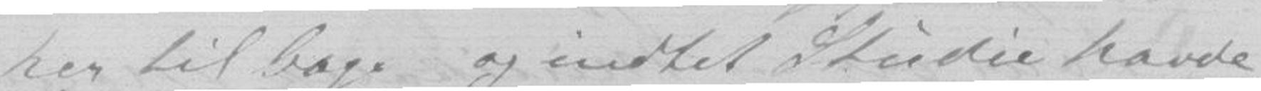her til bage, og intet Studie havde,
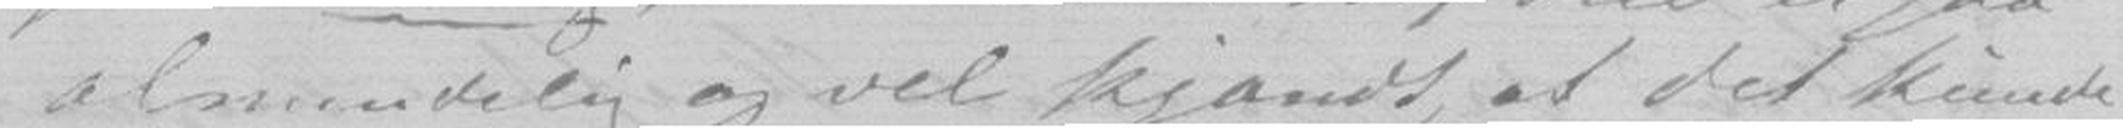almindelig og vel kjændt, at det kunde
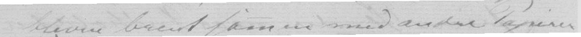bleven brent samen med andre Papirer,
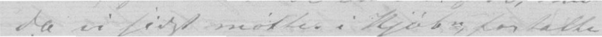da vi sidst møttes i Kjøbn, fortalte
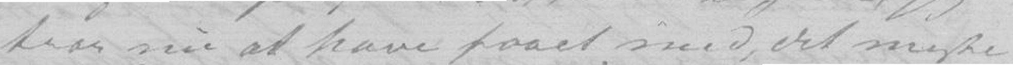jeg tror nu at have faaet med, det meste
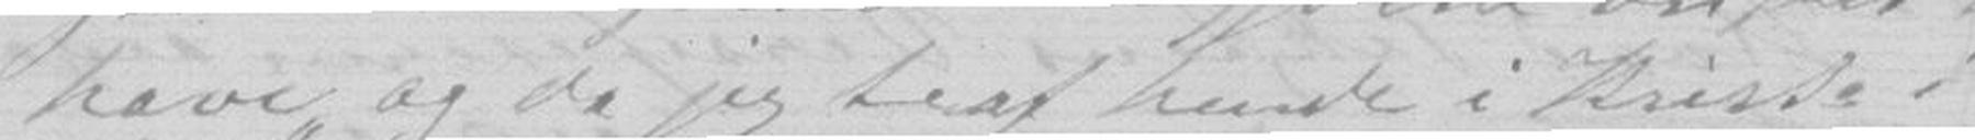have og da jeg traf hende i Krista i
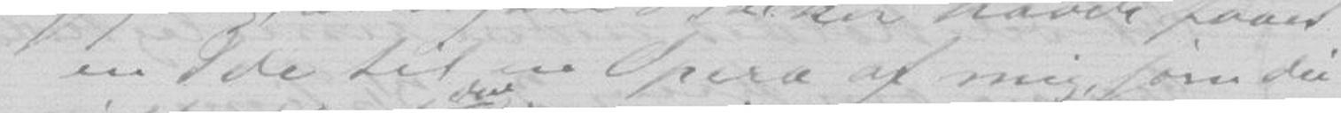en Ide til en Opera af mig, som du
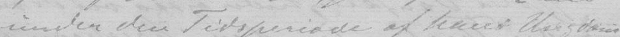under den Tidsperiode af hans Ungdom
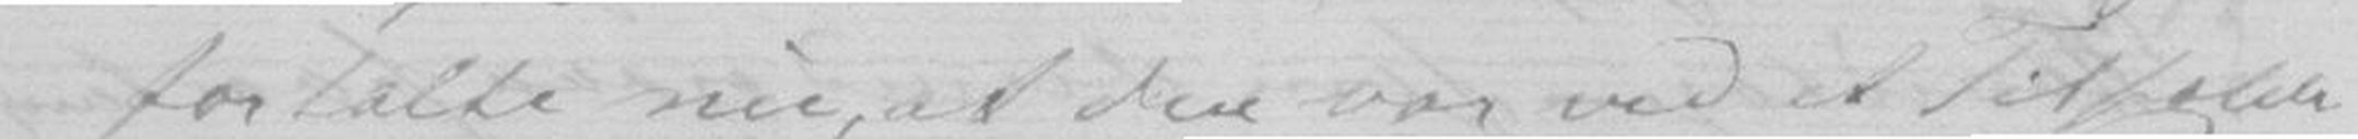fortalte nu, at der var ved et Tilfælde
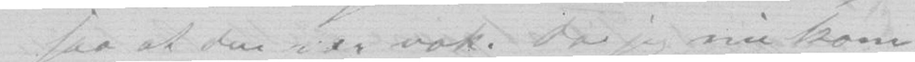saa at den var væk. Da jeg nu kom
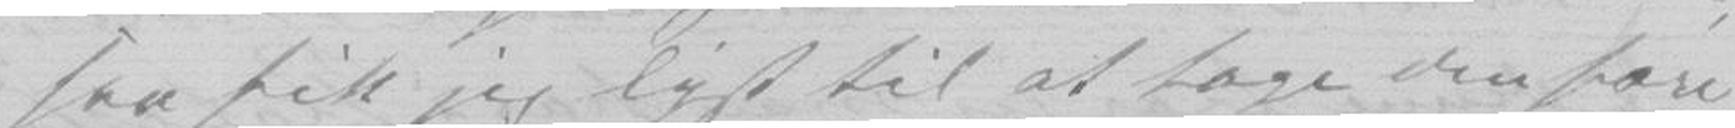saa fik jeg lyst til at tage den fære
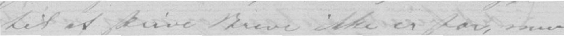til at skrive Breve ikke er stor, men
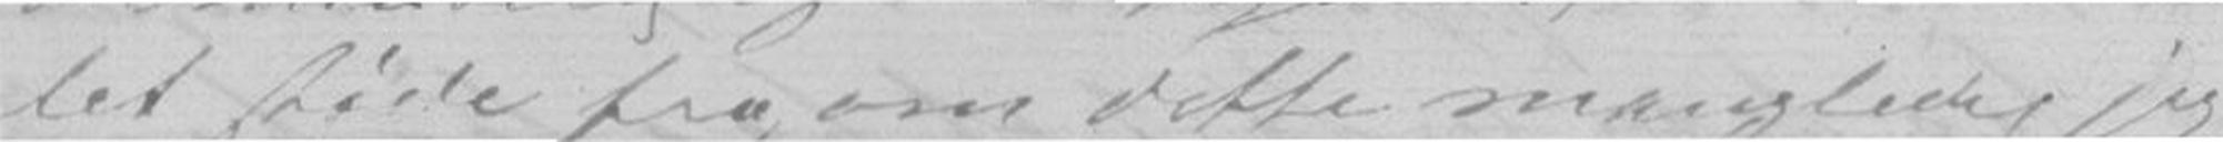let støde fra, om dette manglede,
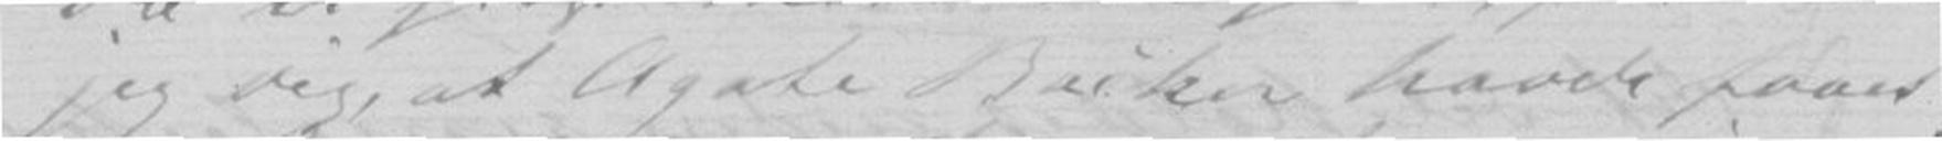jeg dig, at Agate Backer havde faaet
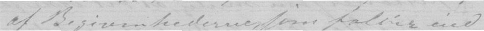af Begivenhederne, som falder ind
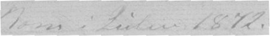Rom i Julen 1872.
